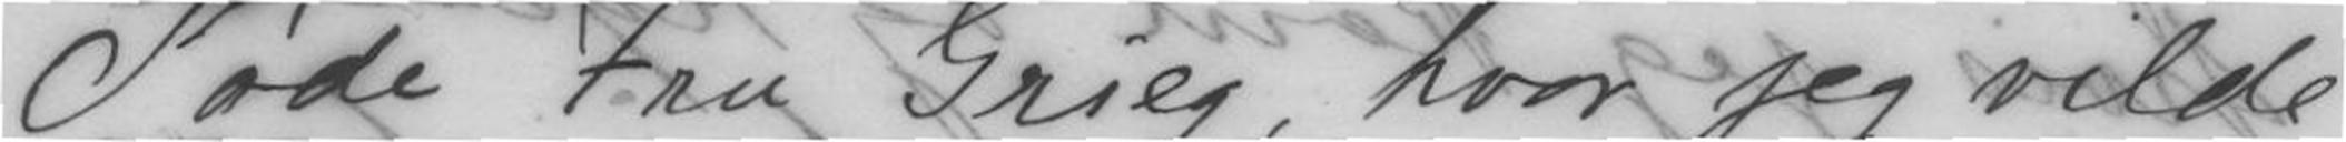Søde Fru Grieg, hvor jeg vilde
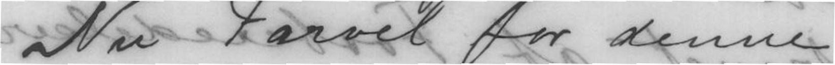Nu Farvel for denne
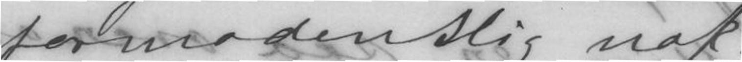formodentlig nok.
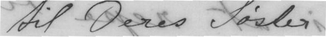til Deres Søster
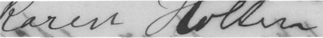Karen Holten
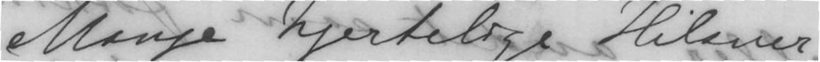Mange hjertelige Hilsner
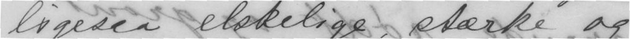ligesaa elskelige, stærke og
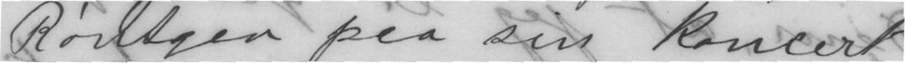Röntgen paa sin koncert
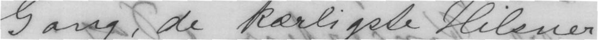Gang, de kærligste Hilsner
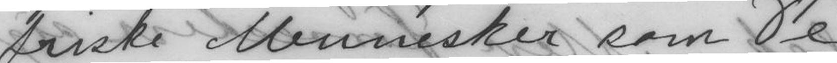friske Mennesker som De
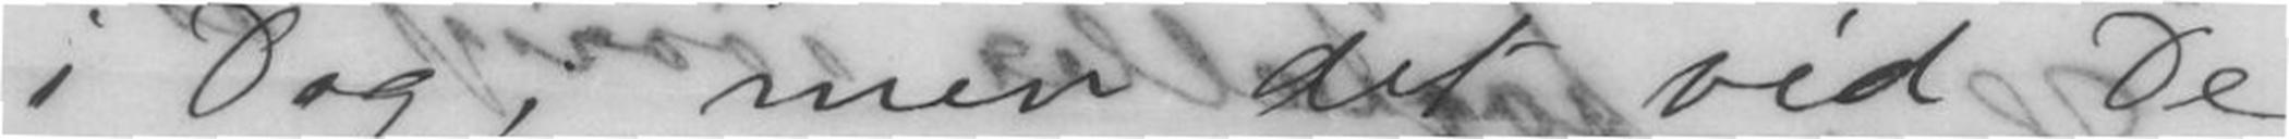i Dag, men det vil De
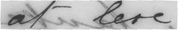at leve
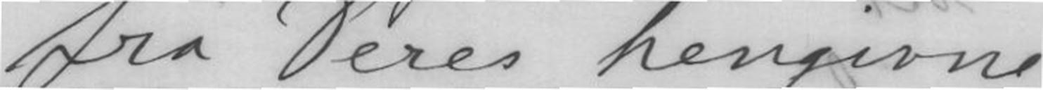fra Deres hengivne
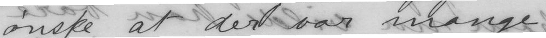ønske at det var mange
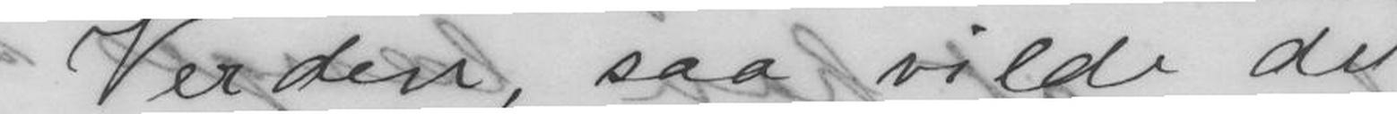i Verden, saa vilde det
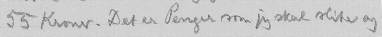55 Kroner. Det er Penger som jeg skal slite og
